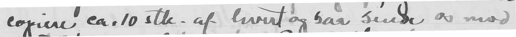copiere ca. 10 stk - af hvert og saa sende os mod
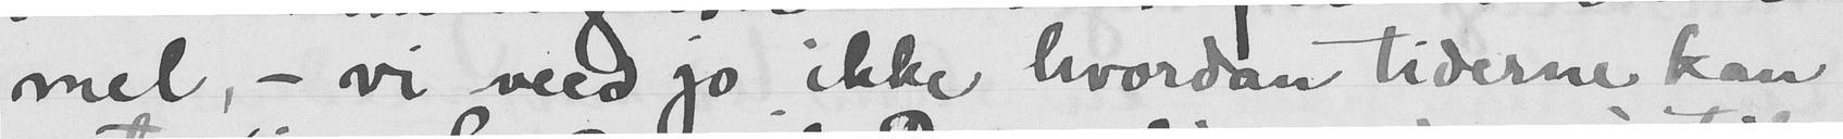mel, - vi veed jo ikke hvordan tiderne kan
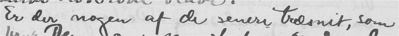Er der nogen af de senere træsnit, som
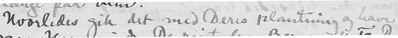Hvorledes gik det med Deres plantning og have
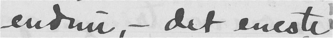endnu, - det eneste
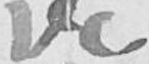vi
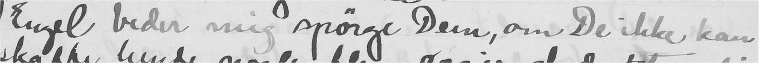Engel beder mig spørge Dem, om De ikke kan
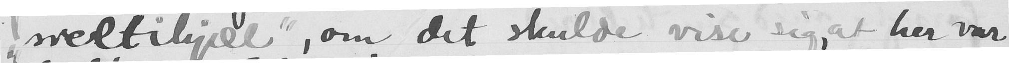"sveltihjæl", om det skulde vise sig, at her var
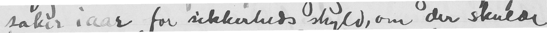saker i aar for sikkerheds skyld, om der skulde
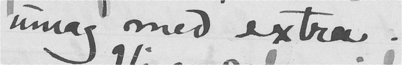umag med extra.
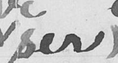ser
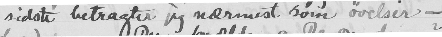sidste betragter jeg nærmest som øvelser -
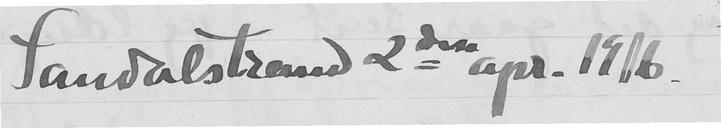Sandalstrand 2den apr. 1916.
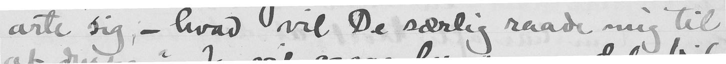arte sig, - hvad vil De særlig raade mig til
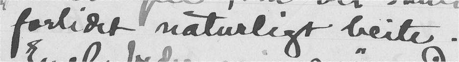forlidet naturligt beite.
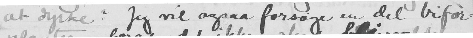at dyrke? Jeg vil ogsaa forsøge en del bifor-
